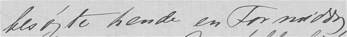besøgte hende en Formiddag
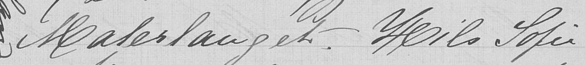Malerlauget. Hils Sofie
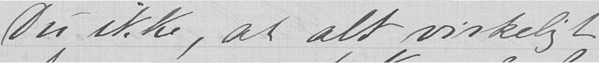Du ikke, at alt virkeligt
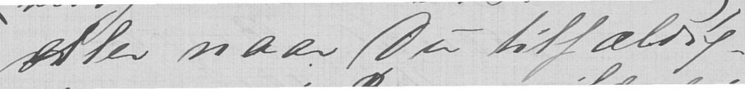atter naar Du tilfældig
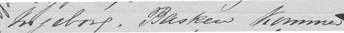lngeborg. Basken kommer
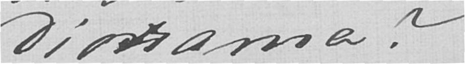Diorama?
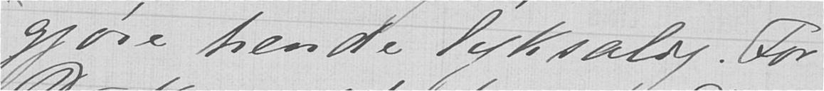gjøre hende lyksalig. For
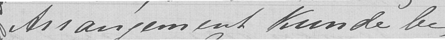Arrangement kunde be-
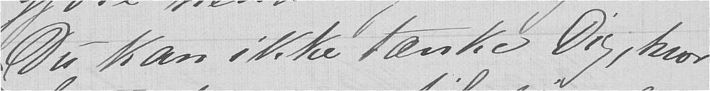Du kan ikke tænke Dig, hvor
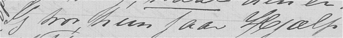Jeg tror hun faar Hjælp
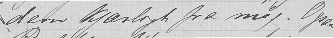dem Kjærligt fra mig. Ogsaa
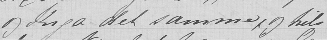og inga det samme, og hils
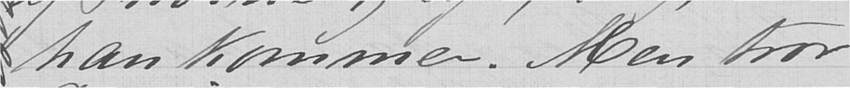han kommer. Men tror
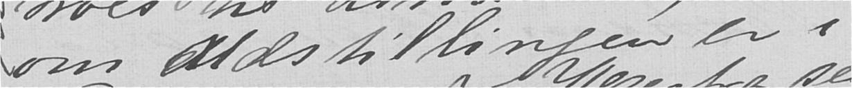om Udstillingen er i
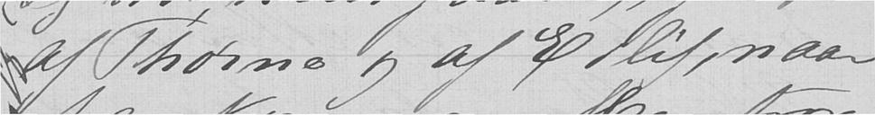af Thørne og af Ellif, naar
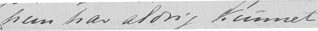hun har aldrig kunnet
